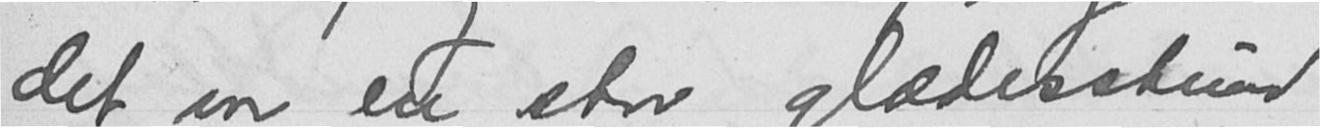det var en stor glædesstund
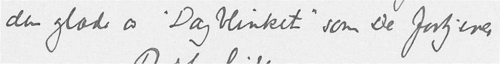den glæde av "Dagblinket" som De fortjener.
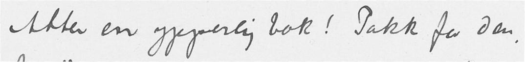Atter en ypperlig bok! Takk for den,
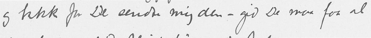og takk for De sendte mig den – gid De maa faa al
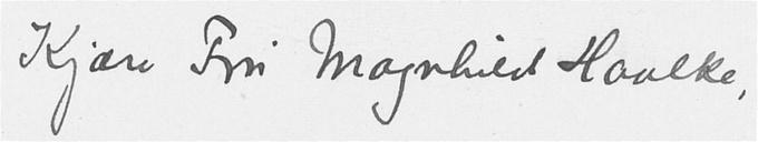Kjære Fru Magnhild Haalke,
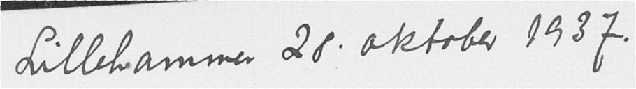Lillehammer 28. oktober 1937.
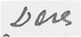Deres
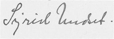Sigrid Undset.
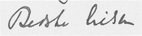Bedste hilsen
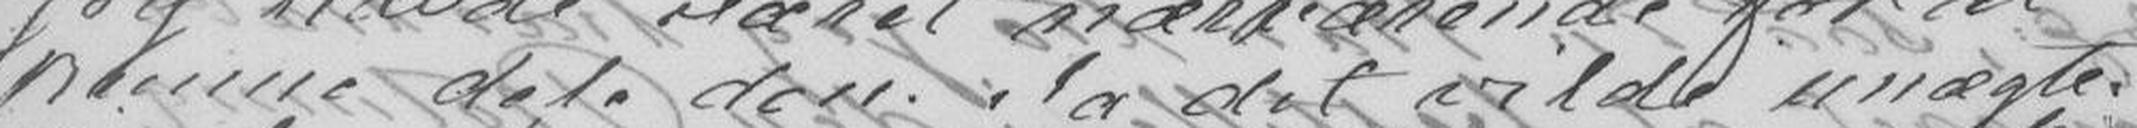kunne dele den. Ja det vilde unægte-
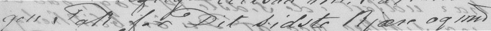gen Tak for Dit sidste kjære og med
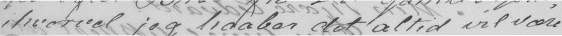hvorvel jeg haaber det altid vil være
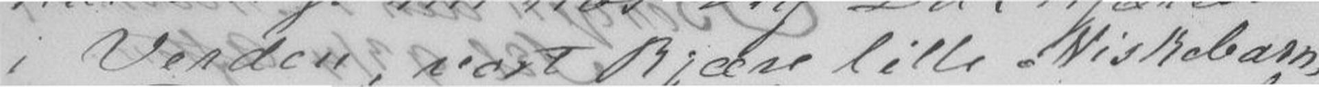i Verden, vort kjære lille Niskebarn,
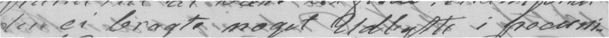den ei bragte noget Udbytte i pecuni-
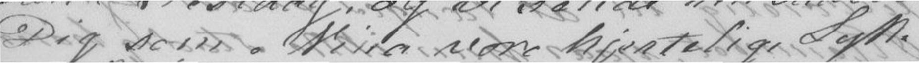Dig som Nina vore hjertelige Lyk-
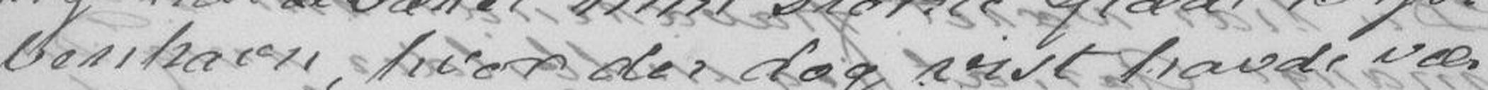benhavn, hvor der dog vist havde væ-
